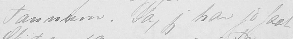Tannum. Ja, jeg har jo faat
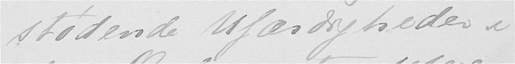stødende Ufærdigheder i
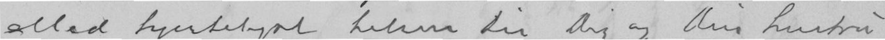Med hjerteligst hilsen til Dig og Din hustru
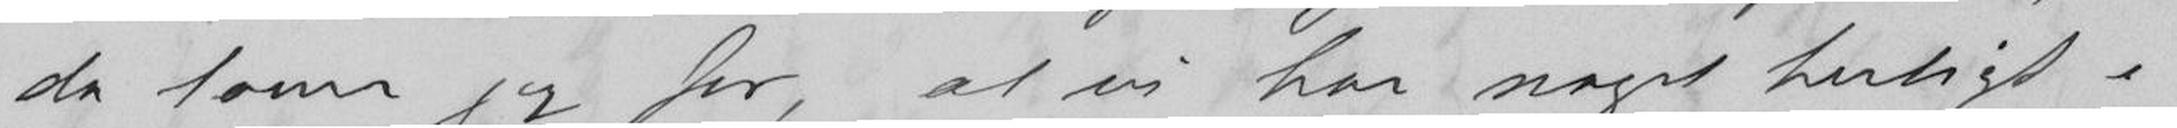da lover jeg for, at vi har noget herligt i
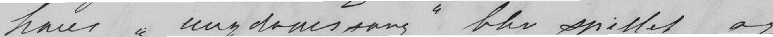hans ungdomssang blir spillet og
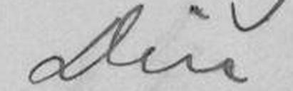Din
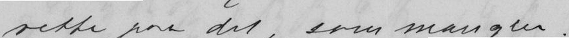rette paa det, som mangler.
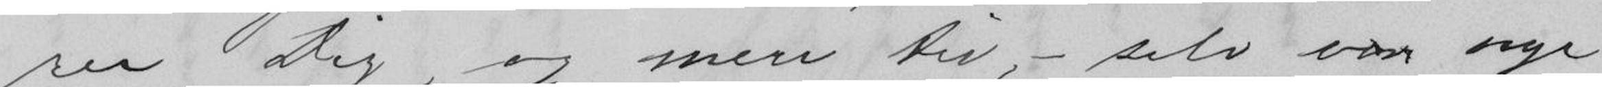rer Dig, og mere tid, - selv vor nye
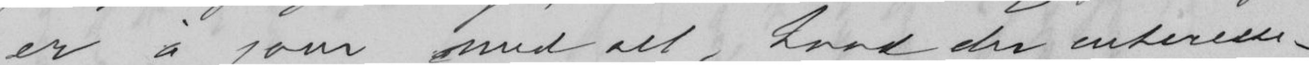er à jour med alt, hvad der interesse-
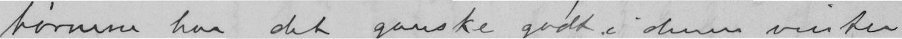børnene har det ganske godt i denne vinter.
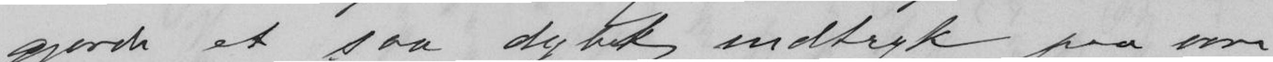gjorde et saa dybt indtryk paa vore
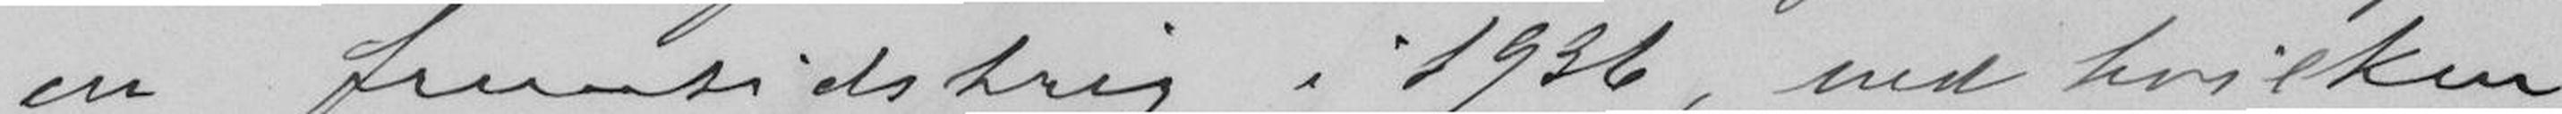en fremtidskrig i 1936, ved hvilken
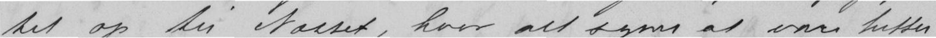tet op til Næsset, hvor alt synes at være lutter
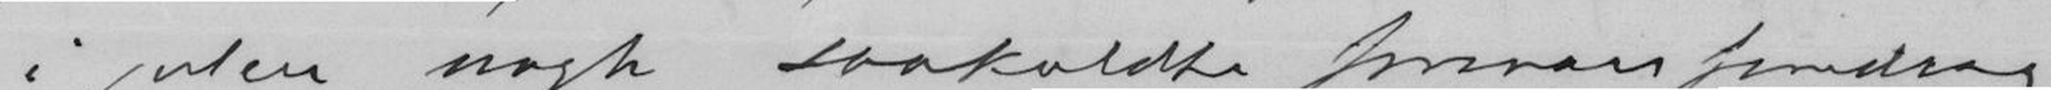i julen nogle saakaldte forsvarsforedrag.
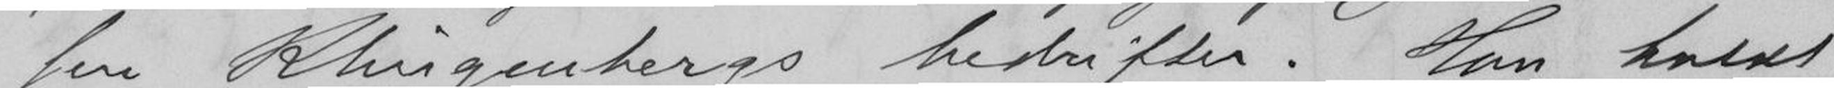sen Klingenbergs bedrifter. Han holdt
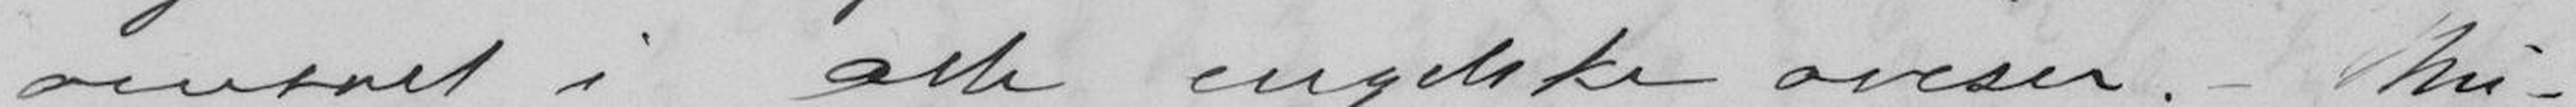omtalt i alle engelske aviser. - Mu-
